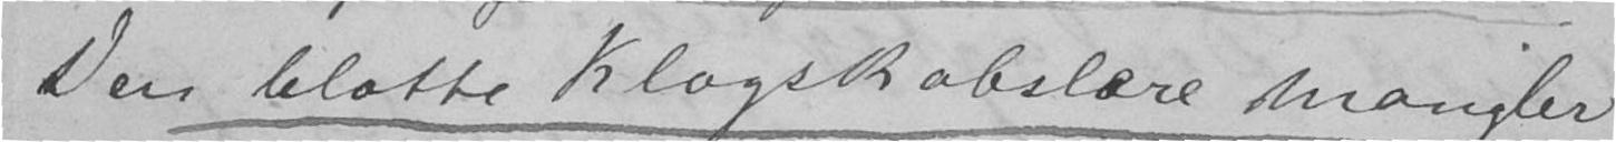Den blotte Klogskabslære mangler
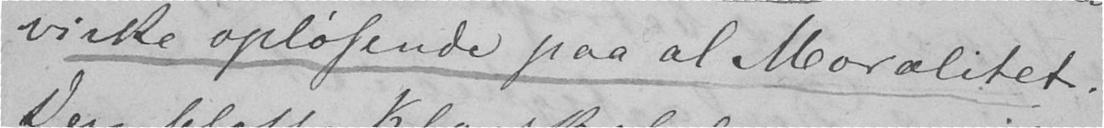virke opløsende paa al Moralitet.
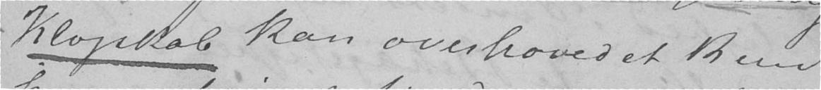Klogskab kan overhovedet kun
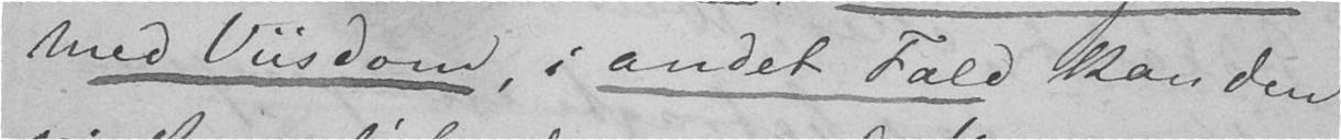med Viisdom, i andet Fald kan den
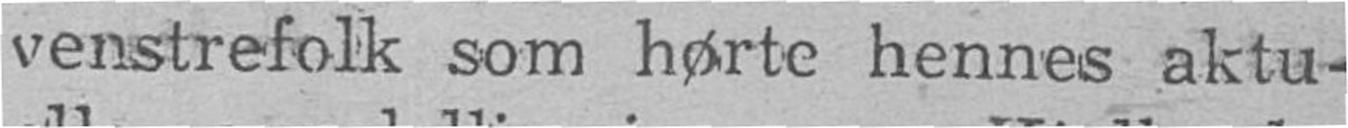venstrefolk som hørte hennes aktu-
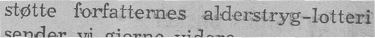støtte forfatternes alderstryg-lotteri
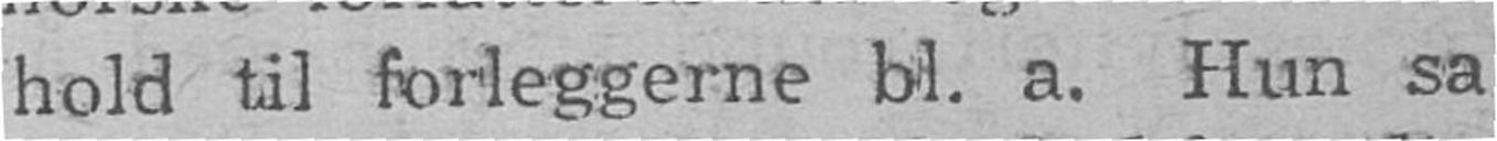hold til forleggerne bl. a. Hun sa
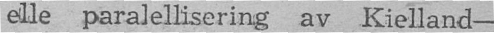elle paralellisering av Kielland -
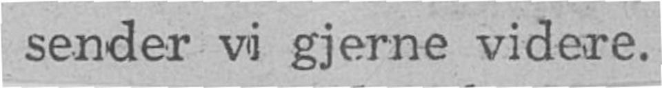sender vi gjerne videre.
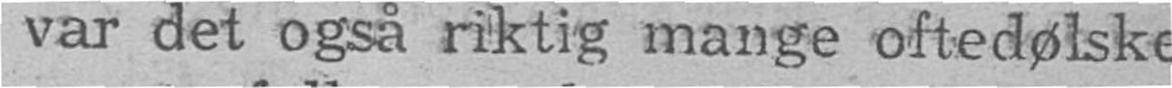var det også riktig mange oftedølske
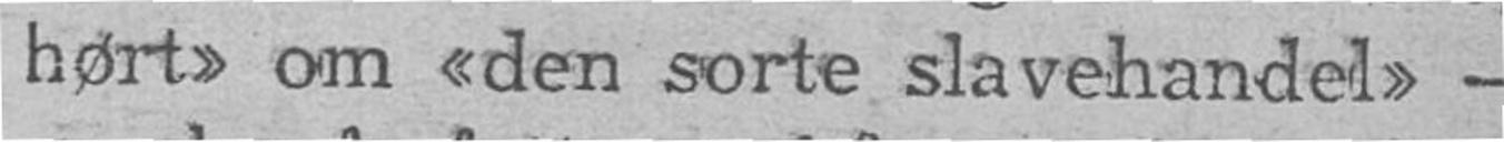hørt» om «den sorte slavehandel» -
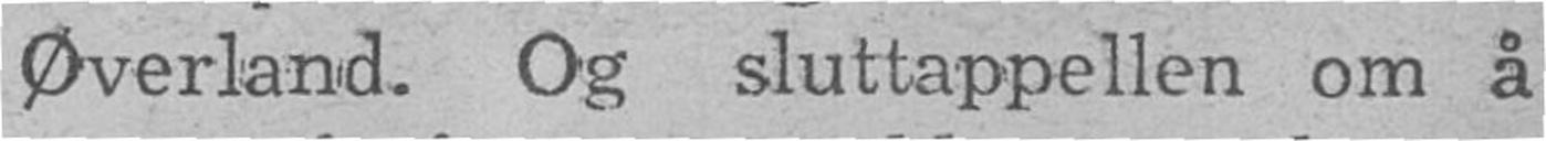Øverland. Og sluttappellen om å
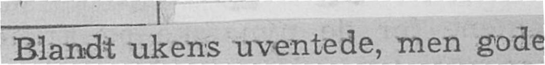Blandt ukens uventede, men gode
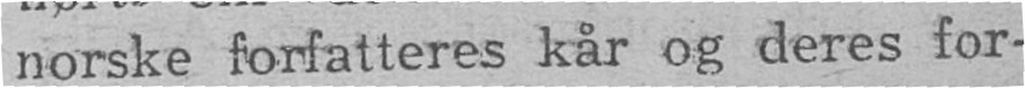norske forfatteres kår og deres for-
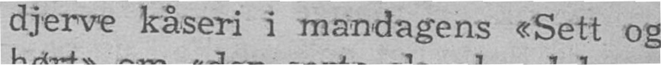djerve kåseri i mandagens «Sett og
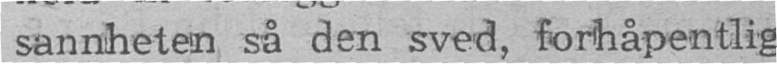sannheten så den sved, forhåpentlig
